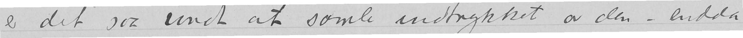er det saa vondt at samle indtrykket av den – endda
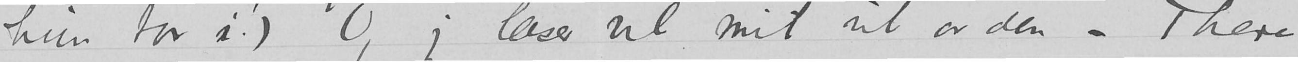hun tar i!) Og jeg læser vel mit ut av den – There
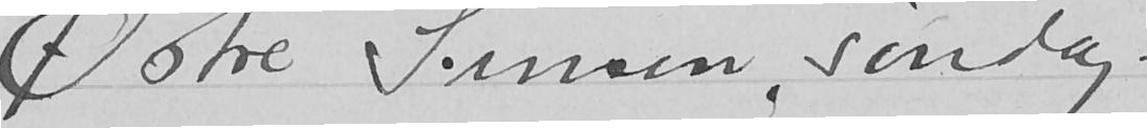Øvre Sinsen, søndag
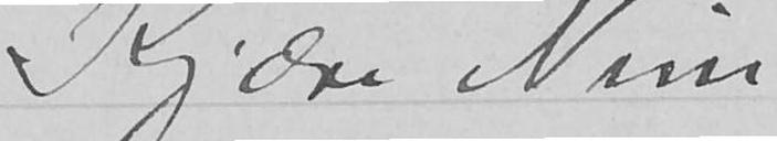Kjære Nini
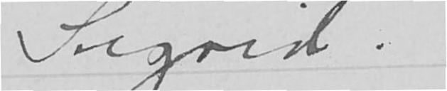Sigrid
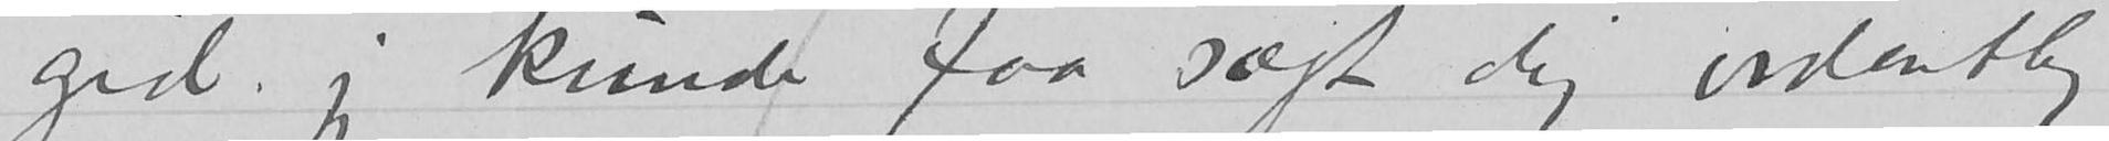gid jeg kunde faa sagt dig ordentlig, hvor god jeg synes boken din er
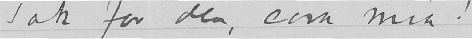Tak for den, cora mia!
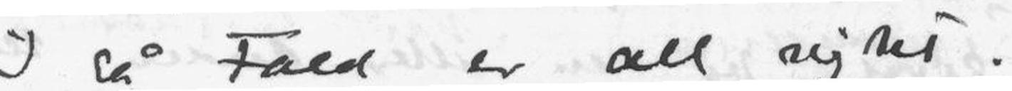I så Fald er all rigtig.
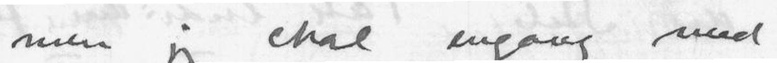men jeg skal engang med
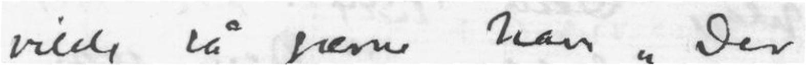vilde så gærne have "Der
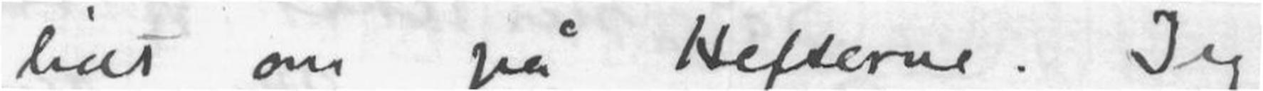lidt om på Hefterne. Jeg
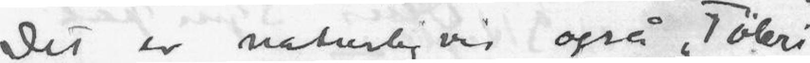Det er naturligvis også "Føleri
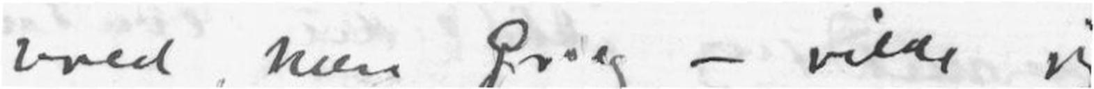vred, men Grieg - vilde jeg
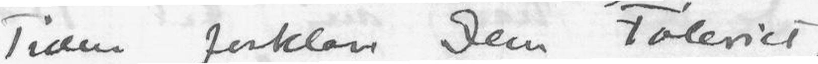Tiden forklare Dem Føleriet.
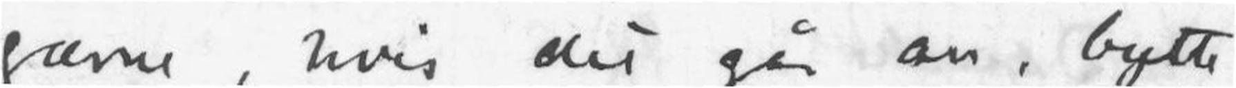gærne, hvis det går an, bytte
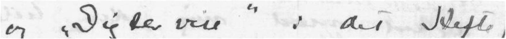og Digter vise i det Hefte
